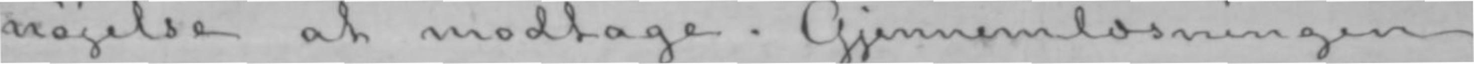nøjelse at modtage. Gjennemlæsningen
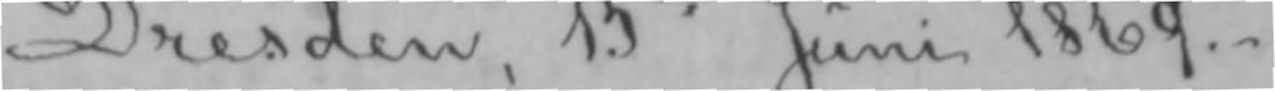Dresden, 15de Juni 1869.-
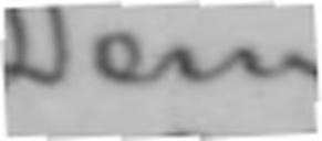Dem.
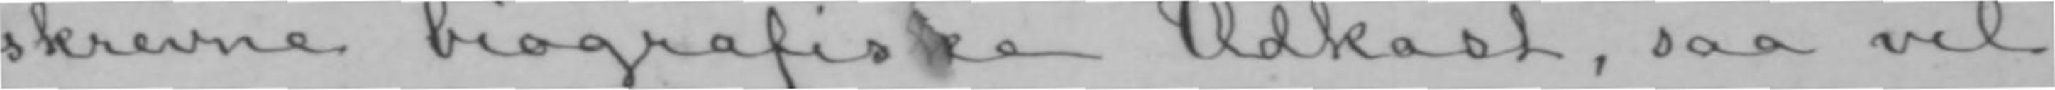skrevne biografiske Udkast, saa vil
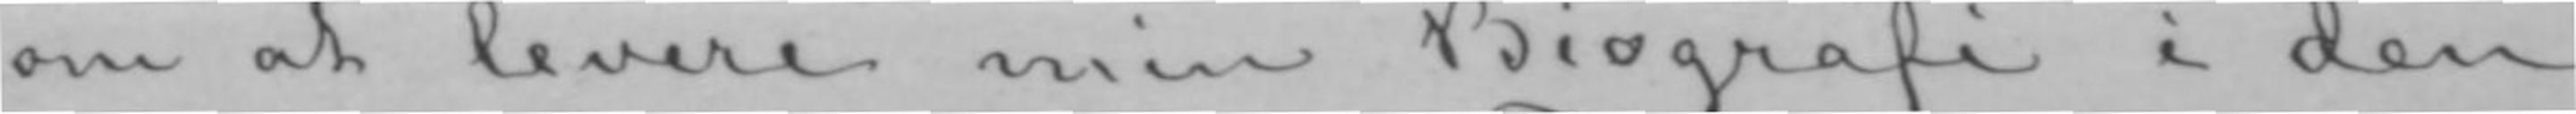om at levere min Biografi i den
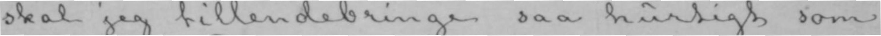skal jeg tillendebringe saa hurtigt som
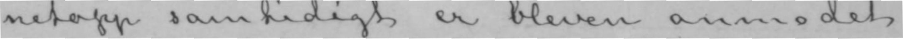netopp samtidigt er bleven anmodet
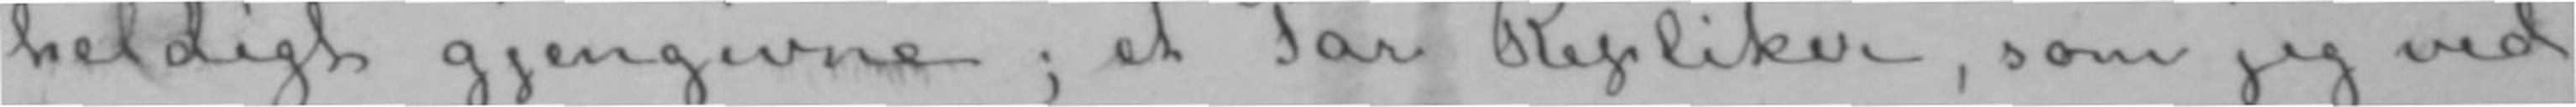heldigt gjengivne; et Par Repliker, som jeg ved
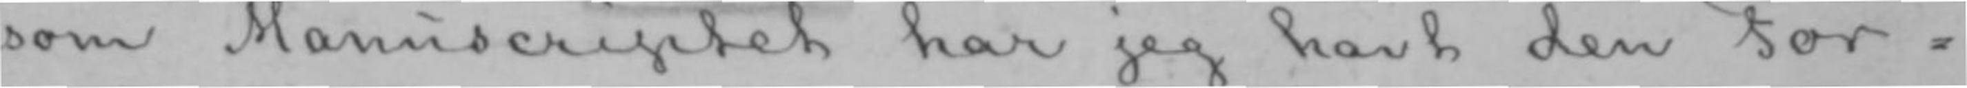som Manuscriptet har jeg havt den For-
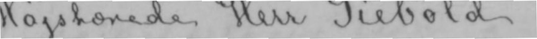Højstærede Herr Siebold!
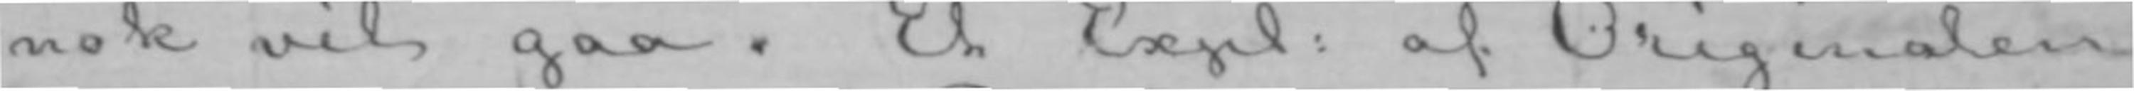nok vil gaa. Et Expl: af Originalen
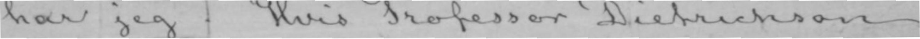har jeg. Hvis Professor Dietrichson
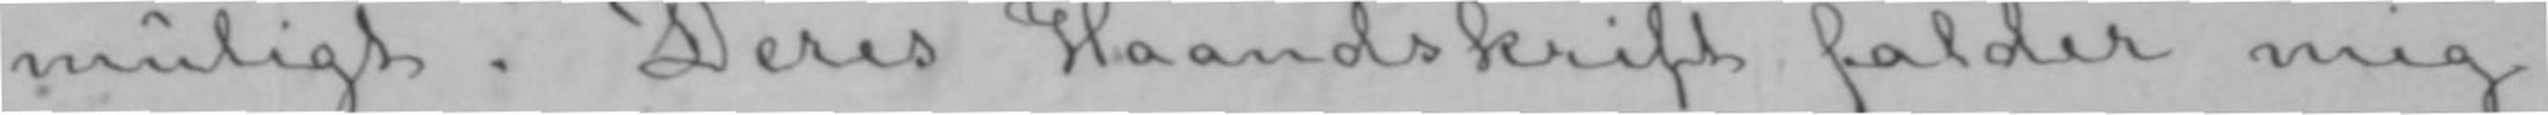muligt. Deres Haandskrift falder mig
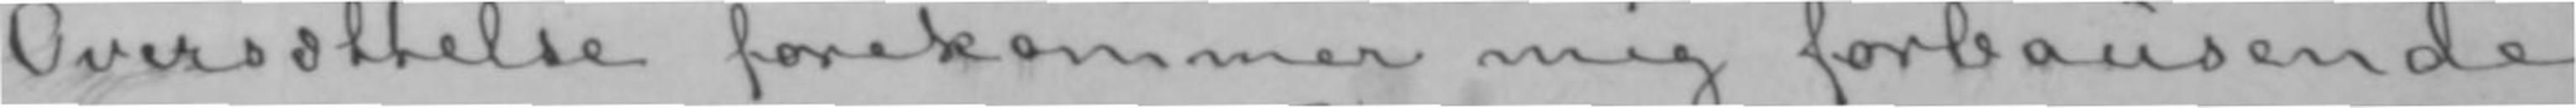Oversættelse forekommer mig forbausende
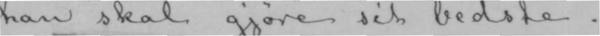han skal gjøre sit bedste.
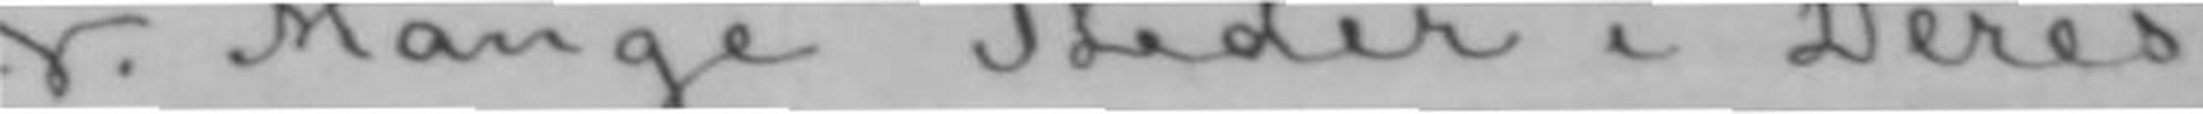Mange Steder i Deres
